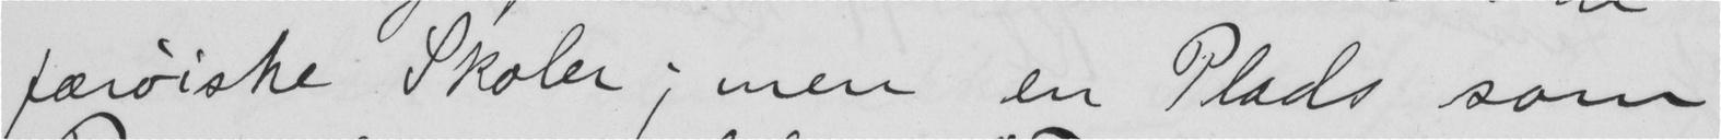færøiske Skoler; men en Plads som
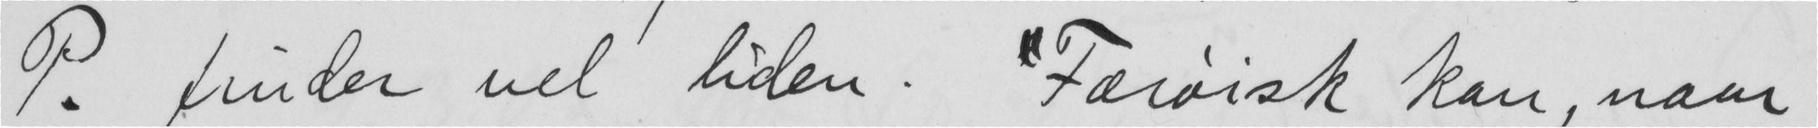P. finder vel liden. "Færøisk kan, naar
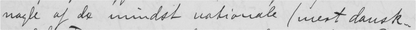nogle af de mindst nationale (mest dansk-
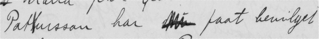Patursson har  faat bevilget
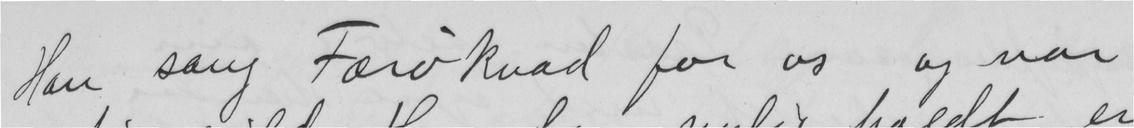Han sang Færøkvad for os og var
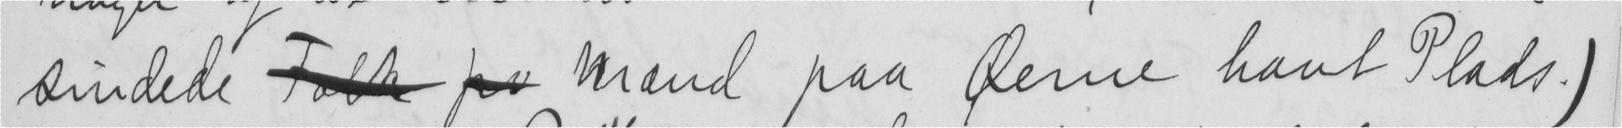sindede  Mænd paa Øerne havt Plads.)
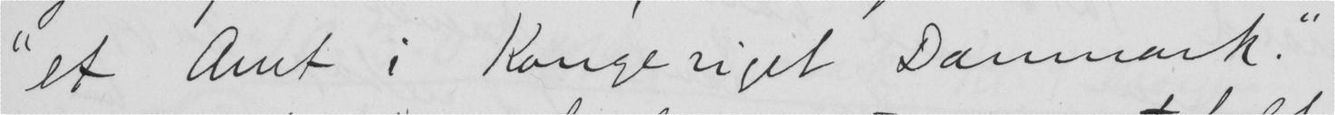"et Amt i Kongeriget Danmark."
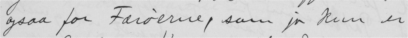ogsaa for Færøerne, som jo kun er
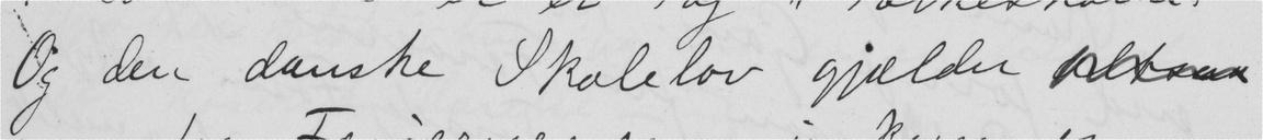Og den danske Skolelov gjælder
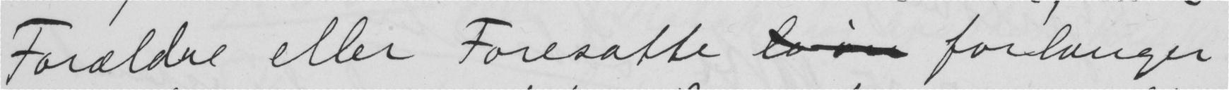Forældre eller Foresatte  forlanger
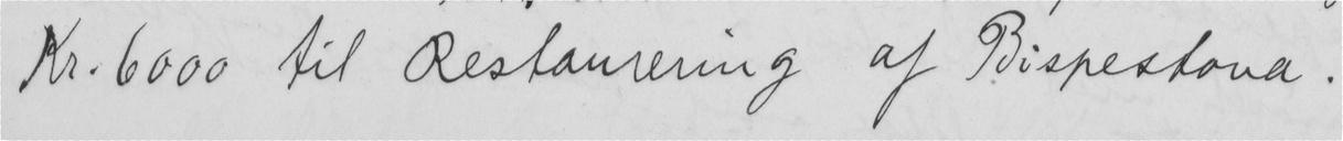Kr.6000 til Restaurering af Bispestova.
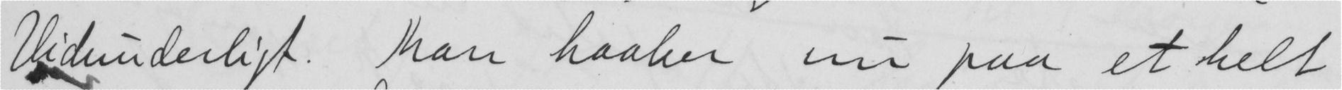Vidunderligt. Man haaber nu paa et helt
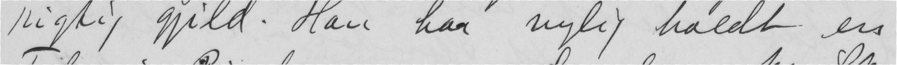rigtig gjild. Han har nylig holdt en
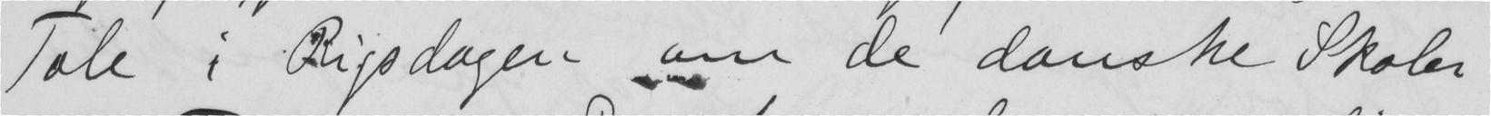Tale i Rigsdagen om de danske Skoler
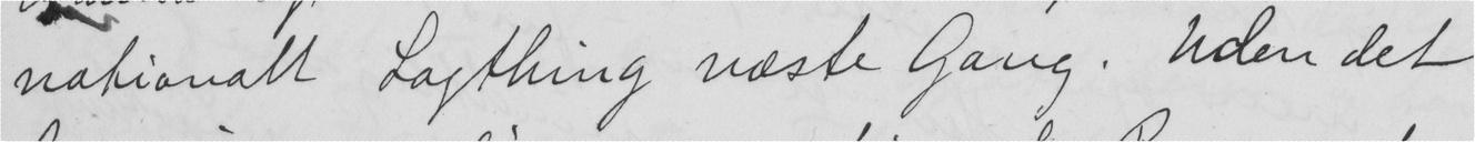nationalt Lagthing næste Gang. Uden det
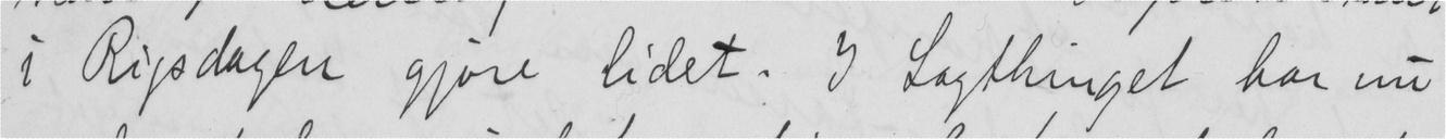i Rigsdagen gjøre lidet. I Lagthinget har nu
